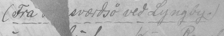(Fra sværdsø ved Lyngby.)
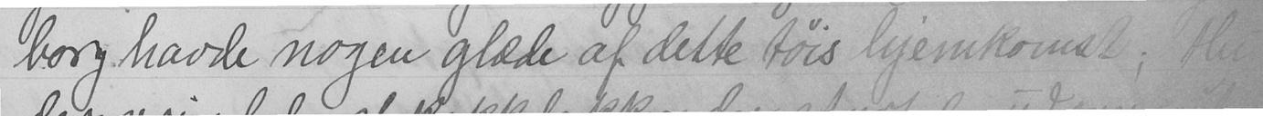borg havde nogen glæde af dette tøis hjemkomst; thi
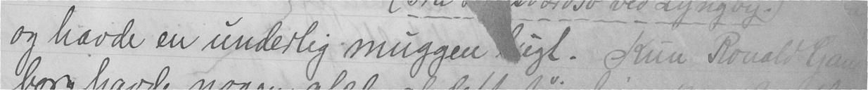og havde en underlig muggen lugt. Kun Ronald, Gam-
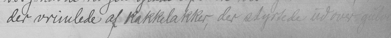der vrimlede af kakkelakker, der styrtede ud over gulvet
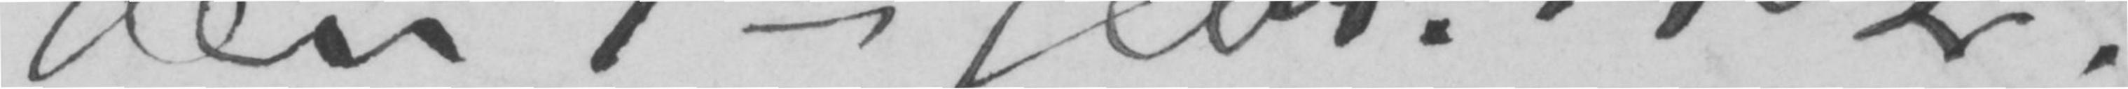den 9de febr. 1902.
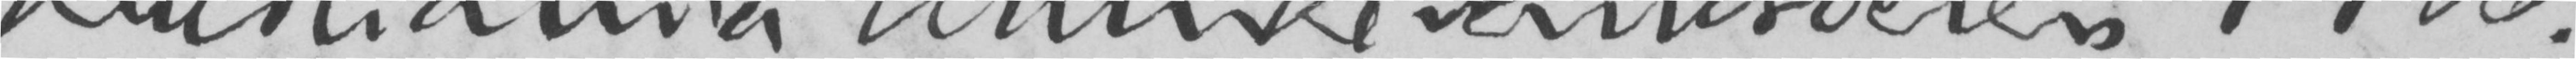Kristiania, Munkedamsveien 44 B.,
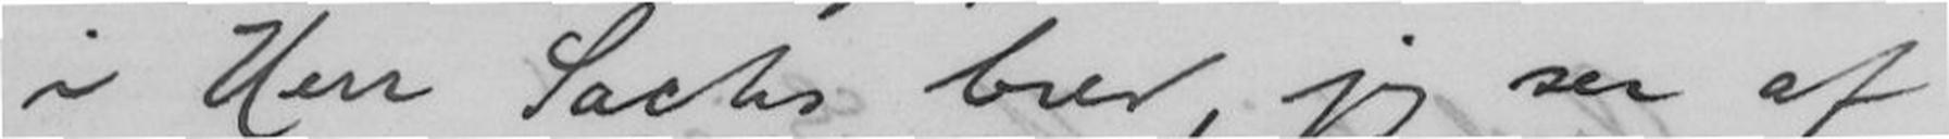i Herr Sachs brev, jeg ser af
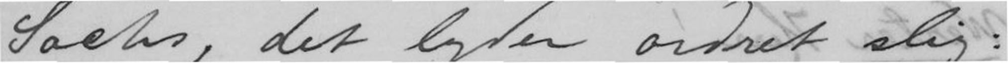Sachs, det lyder ordret slig:
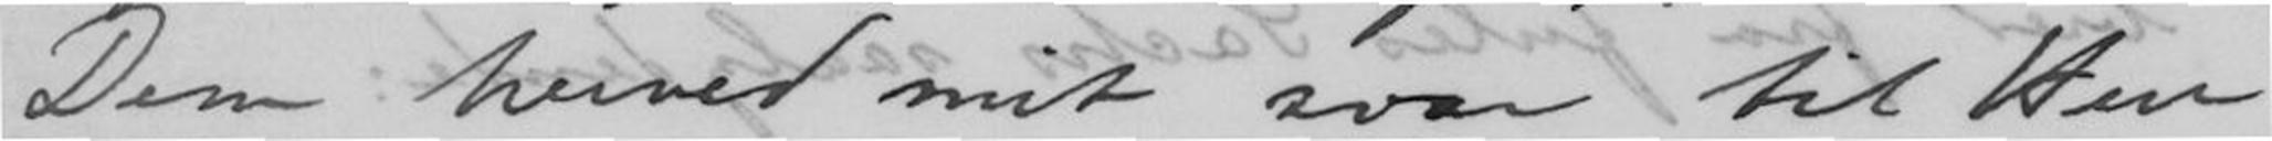Dem herved mit svar til Herr
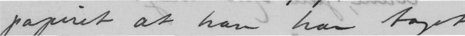papiret at han har taget
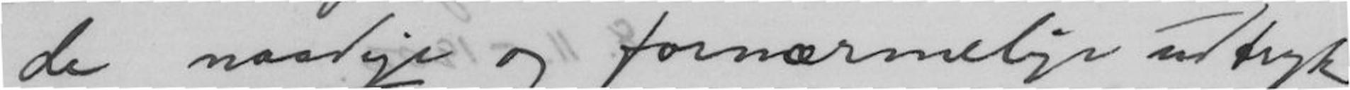de naadige og fornærmelige udtryk
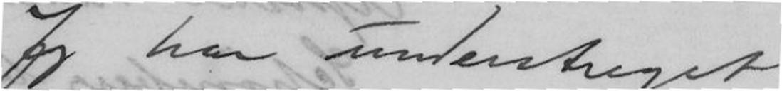Jeg har understreget
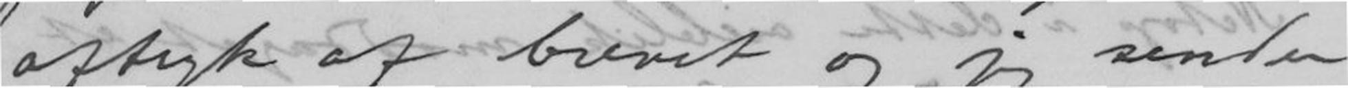aftryk af brevet og jeg sender
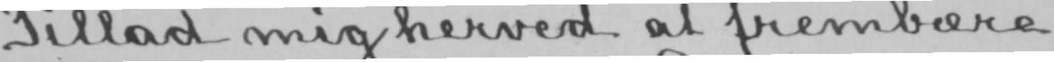Tillad mig herved at frembære
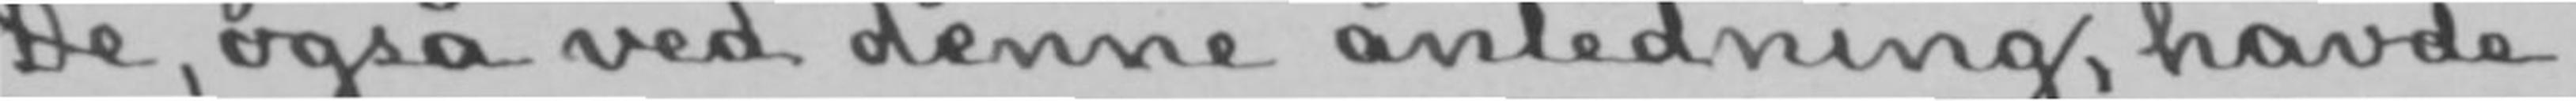De, også ved denne anledning, havde
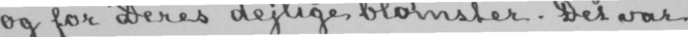og for Deres dejlige blomster. Det var
